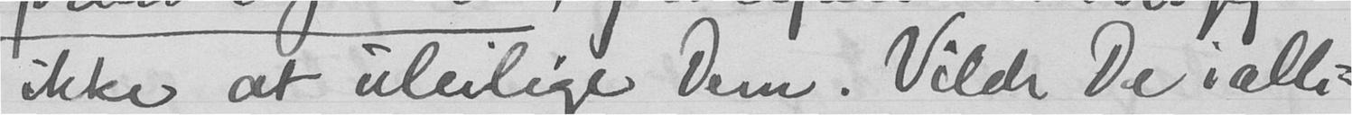ikke at uleilige Dem. Vilde De ialle-
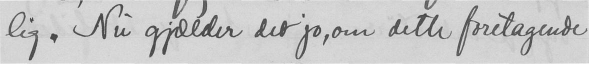lig. Nu gjælder det jo, om dette foretagende
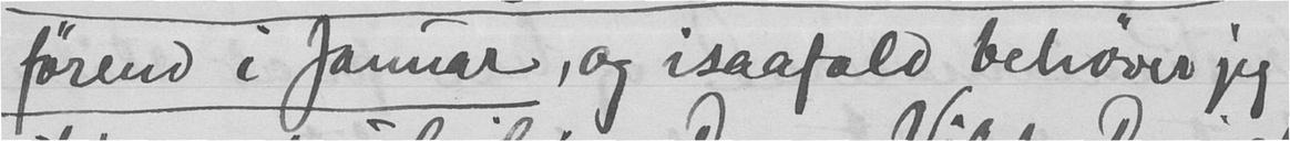førend i Januar, og isaafald behøver jeg
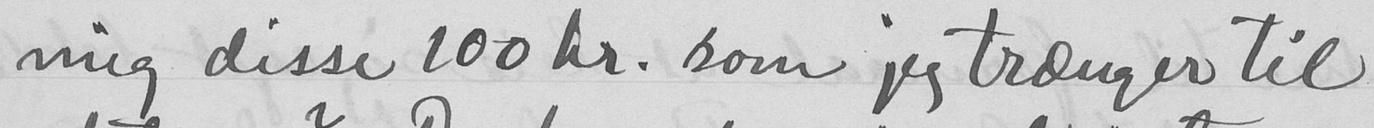mig disse 100 kr. som jeg trænger til
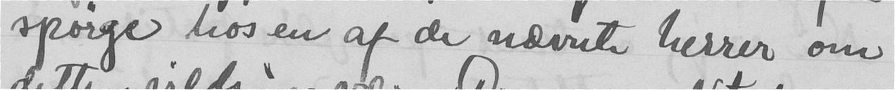spørge hos en af de nævnte herrer om
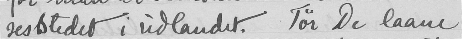sesstedet i udlandet. Tør De laane
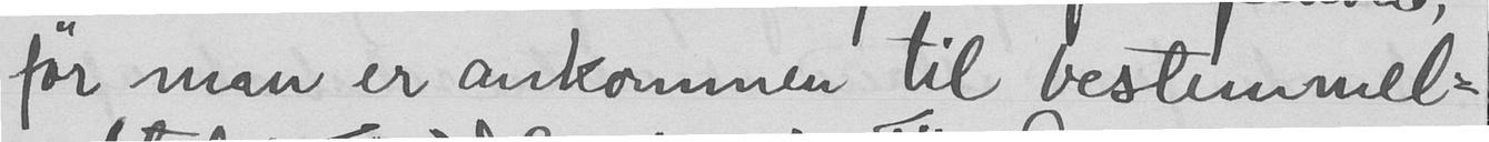før man er ankommen til bestemmel-
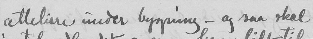atteliere under bygning, - og saa skal
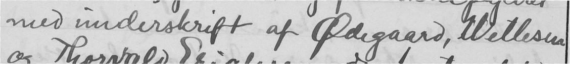med underskrift af Ødegaard, Wetlesen
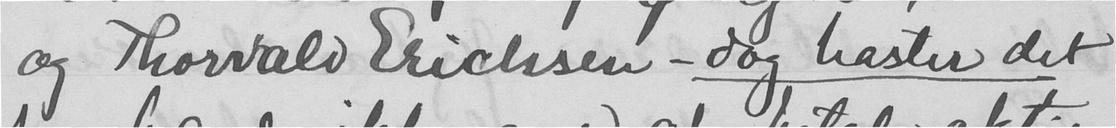og Thorvald Erichsen - dog haster det
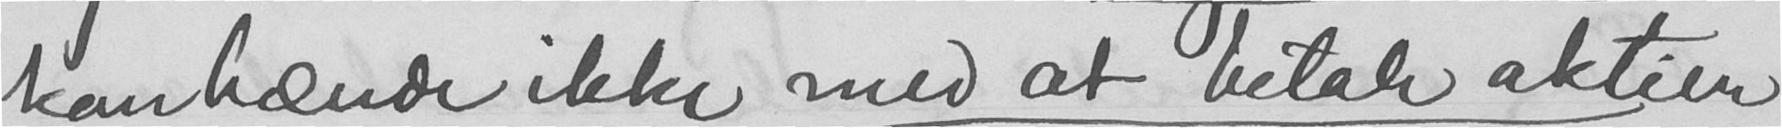kan hænde ikke med at betale aktien
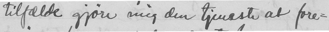tilfælde gjøre mig den tjeneste at fore-
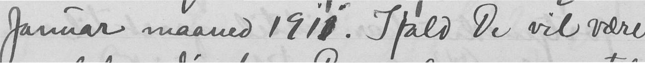Januar maaned 1918. Ifald De vil være
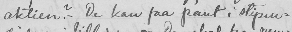aktien? - De kan faa pant i stipen-
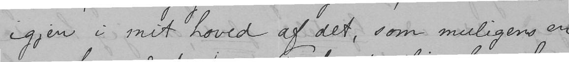igjen i mit hoved af det, som muligens en-
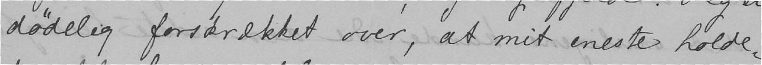dødelig forskrækket over, at mit eneste holde-
